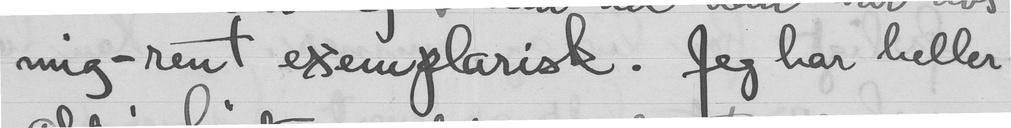mig - rent exemplarisk. Jeg har heller
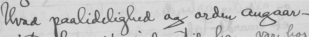Hvad paalidelighed og orden angaar -
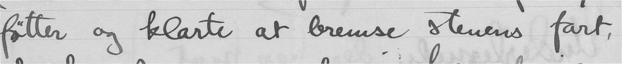føtter og klarte at bremse stenens fart,
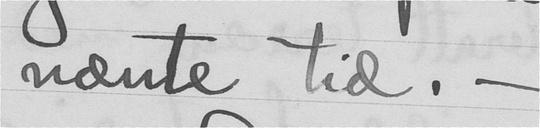nænte tid. -
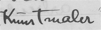"Kunstmaler"
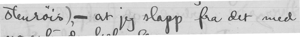stensøis), - at jeg slapp fra det med
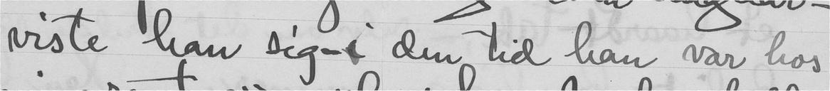viste han sig - i den tid han var hos
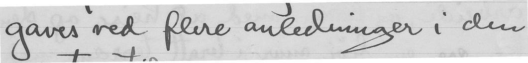gaves ved flere anledninger i den
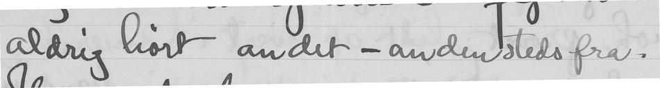aldrig hørt an det - andenstedsfra.
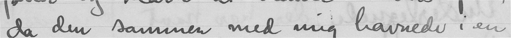da den sammen med mig havnede i en
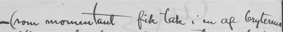- (som momentant fik tak i en af bryterens
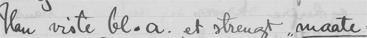Han viste bl. a. et strengt "maate-
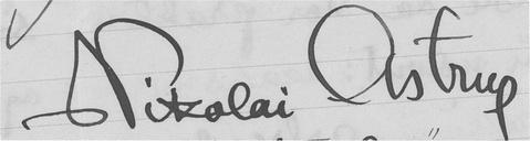Nikolai Astrup
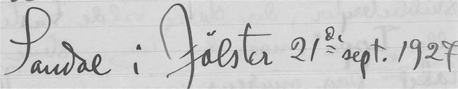Sandal i Jølster 21te sept. 1927
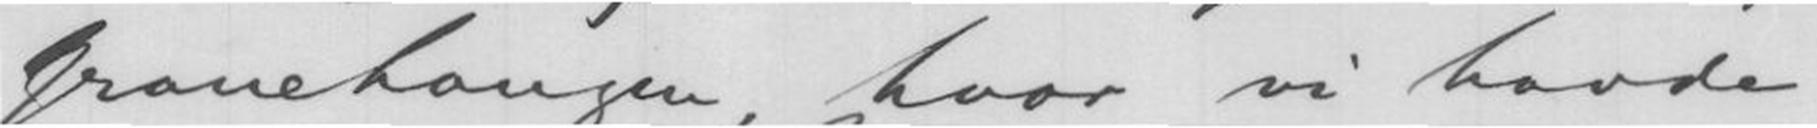Granehaugen ?Granetangen?, hvor vi havde
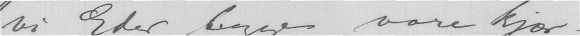vi Eder begge vore kjær-
